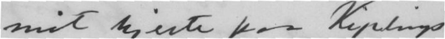mit hjærte paa Kiplings
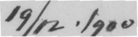19/12. 1900
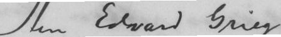Herr Edward Grieg
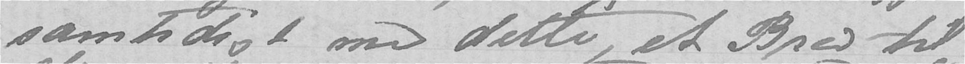samtidigt med dette, et Brev til
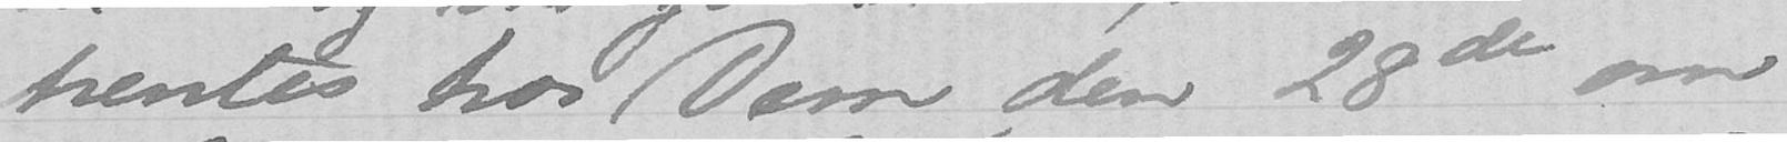hentes hos Dem den 28de om
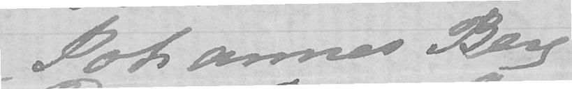Johannes Berg
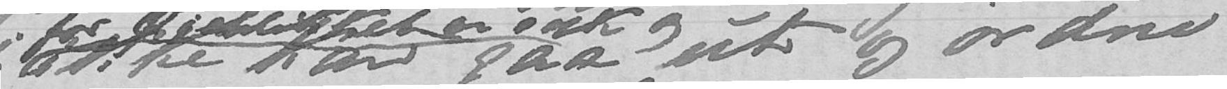ikke kan gaa ut og ordne
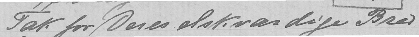Tak for Deres elskværdige Brev
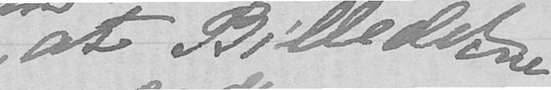at Billedetene
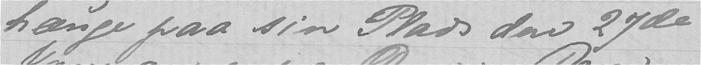hænge paa sin Plads den 27de
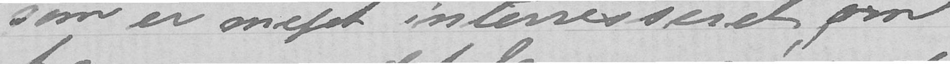som er meget interresseret, om
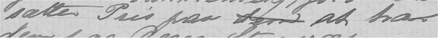sætter Pris paa dem at har
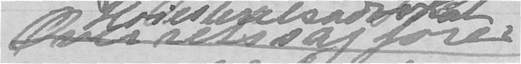Overretssagfører
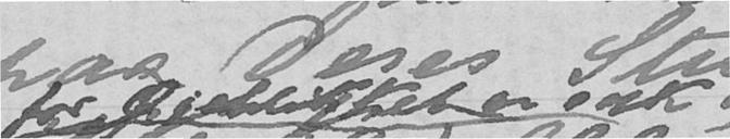for Øieblikket er syk og
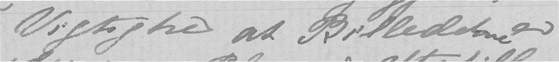Vigtighed at Billedetene er
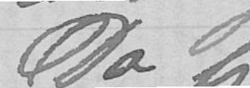Da jeg
